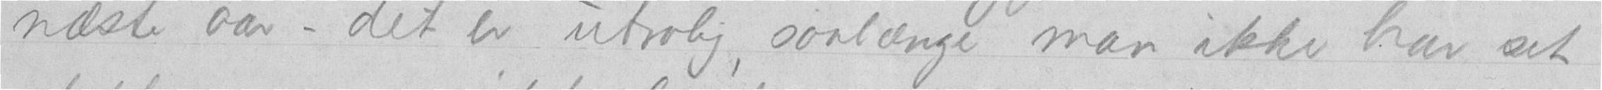næste aar – det er utrolig, saalænge man ikke har set
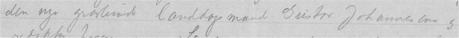den nye gravlund landdagsmand Gustav Johannesens og
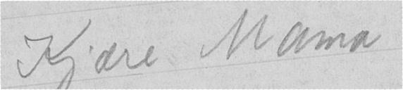Kjære Mama
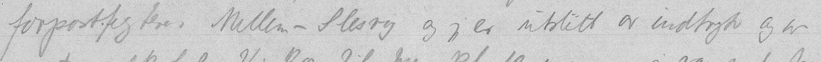forpostfegtere i Mellem-Slesvig og jeg er utslitt av indtryk og av
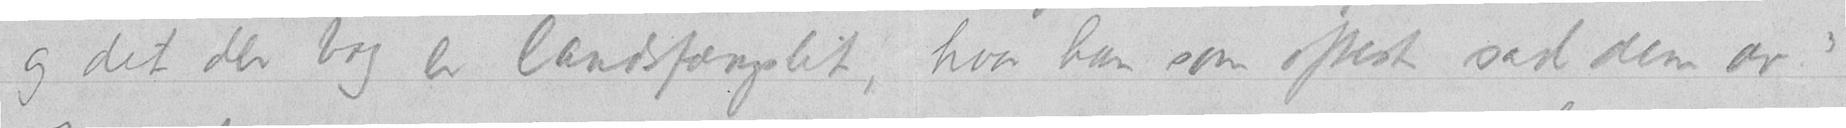og det der bag er landsfængslet, hvor han som oftest sad dem av."
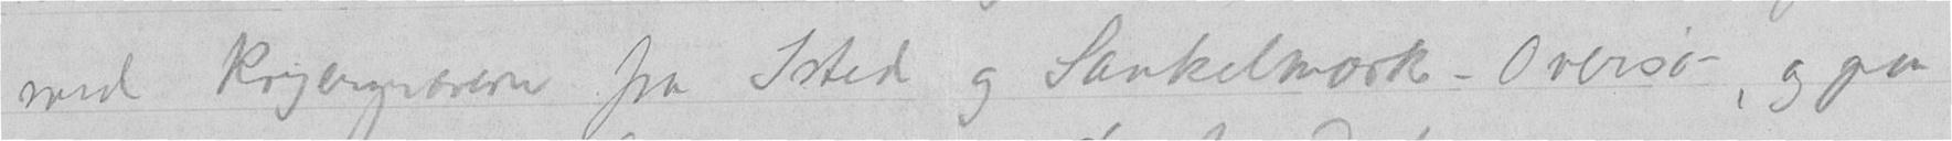med krigergraverne fra Isted og Sankelmark – Oversø –, og paa
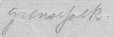grænsefolk.
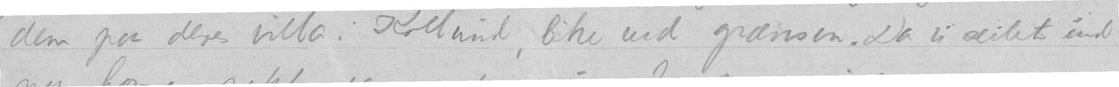dem paa deres villa i Kollund, like ved grænsen. Da vi seilet ind
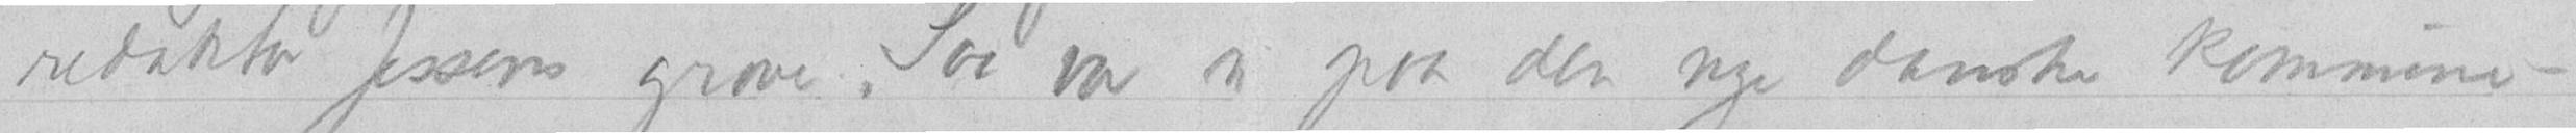redaktør Jessens grave. Saa var vi paa den nye danske kommune-
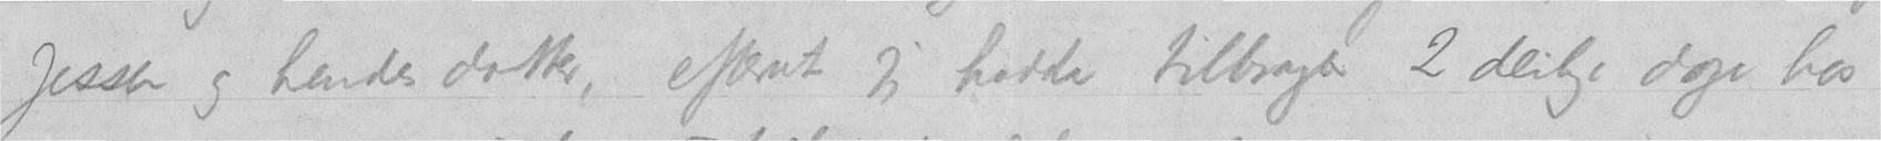Jessen og hendes datter, efterat jeg hadde tilbragt 2 deilige dage hos
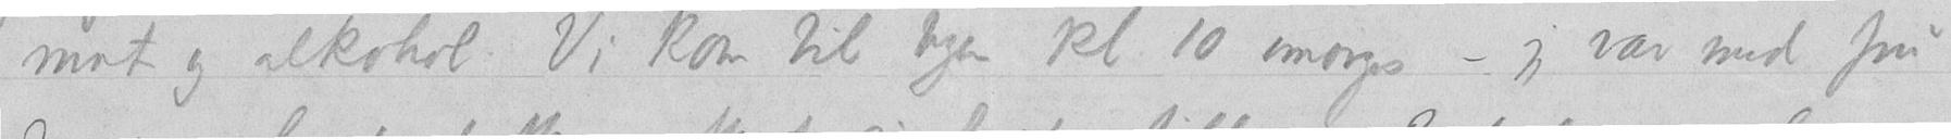mat og alkohol. Vi kom til byen kl 10 imorges – jeg var med fru
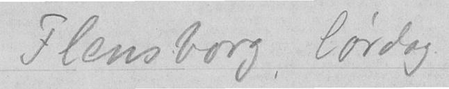Flensborg, lørdag
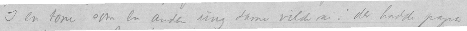I en tone som en anden ung dame vilde si: "der hadde papa
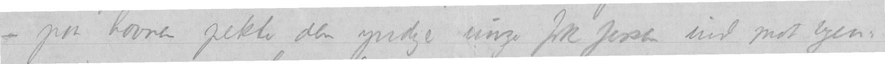paa havnen pekte den yndige unge frk Jessen ind mot byen:
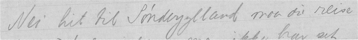Nei hit til Sønderjylland maa du reise
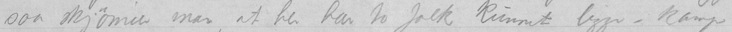saa skjønner man, at her har to folk kunnet ligge i kamp
